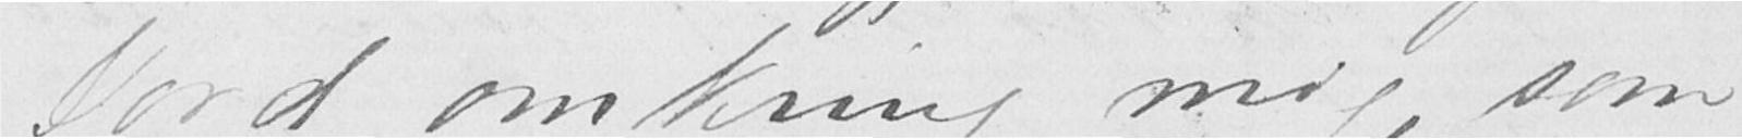Jord omkring mig, som
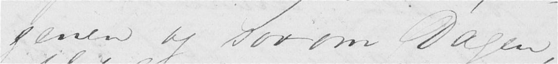genen og sov om Dagen,
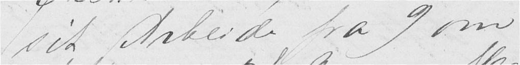set Arbeide fra 9 om
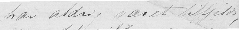har aldrig været tilfjelds,
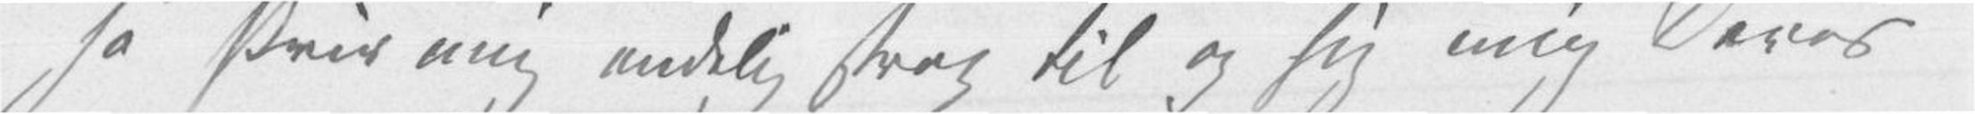så skriv mig endelig strax til og sig mig Deres
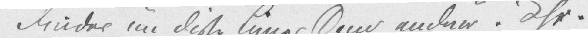Finder nu disse Linier Dem endnu i Chr.
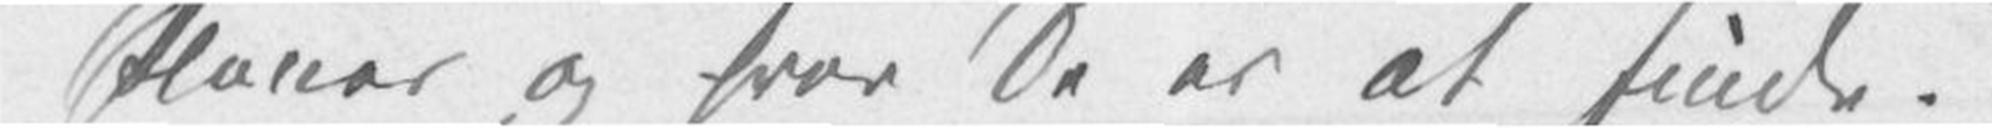Planer og hvor De er at finde.
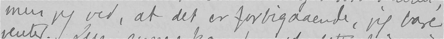men jeg ved, at det er forbigaaende, jeg bare
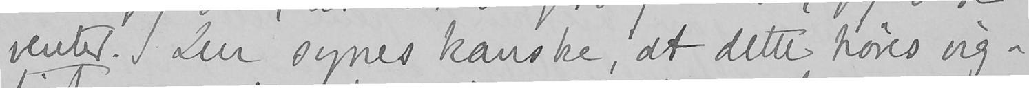venter. Du synes kanske, at dette høres urig-
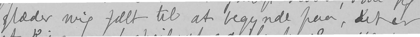glæder mig fælt til at begynde paa, det er
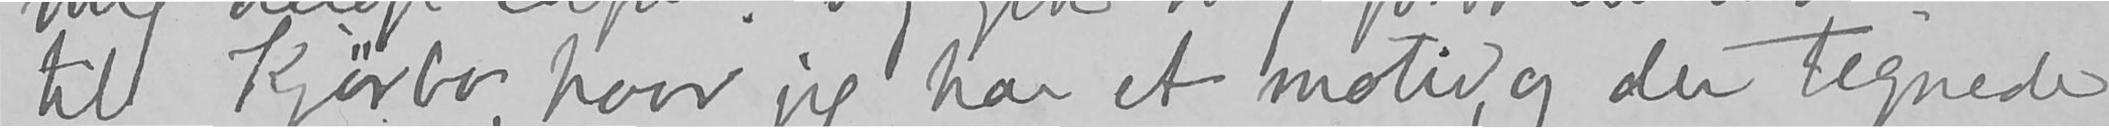til Kjørbo, hvor jeg har et motiv, og der tegnede
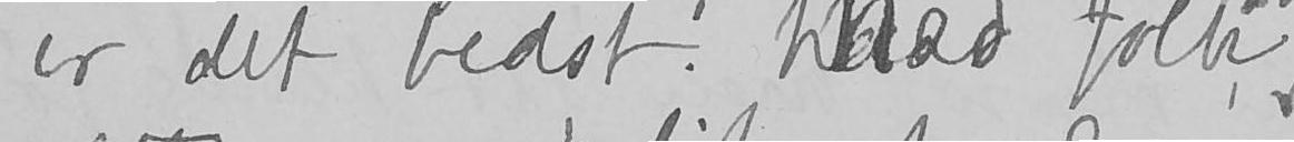er det bedst. Med folk
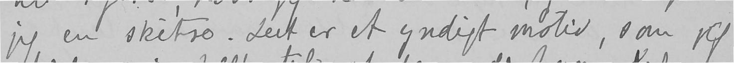jeg en skitse. Det er et yndigt motiv, som jeg
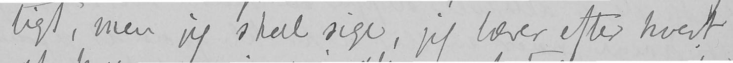tigt, men jeg skal sige, jeg lærer efter hvert
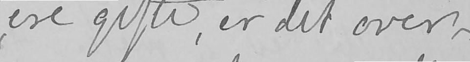ere gifte, er det over
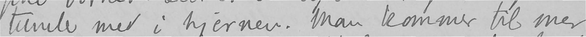tumle med i hjernen. Man kommer til mer
